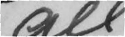all
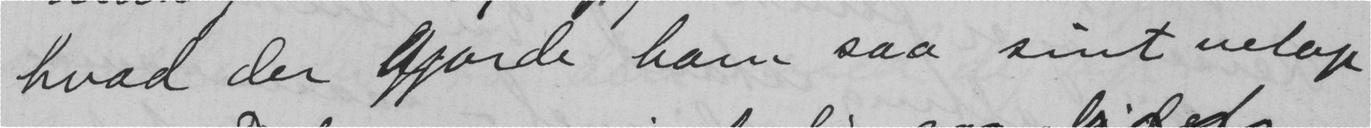hvad der gjorde ham saa sint nelop
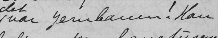var jernbanen! Han
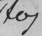tog
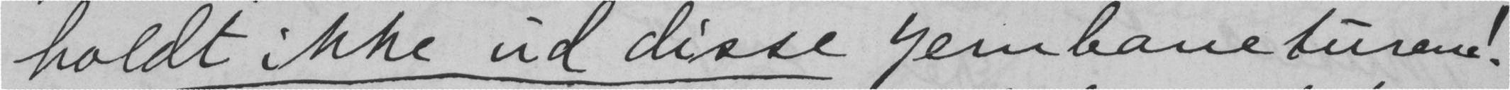holdt ikke ud disse jernbaneturene!
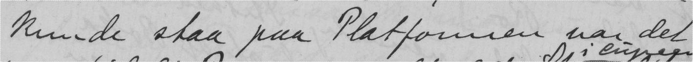kunde staa paa Platformen var det
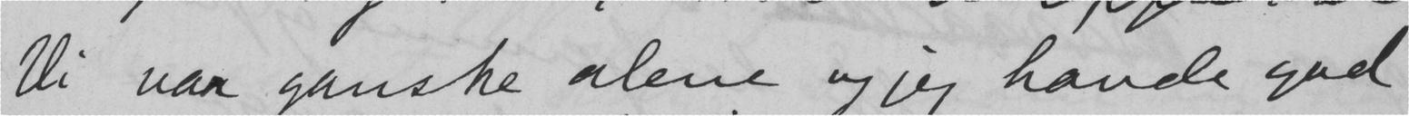Vi var ganske alene og jeg havde god
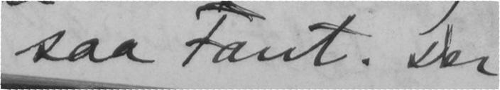saa Fant. Der
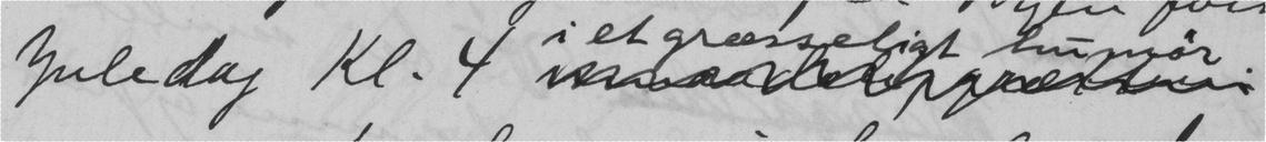Juledag Kl. 4
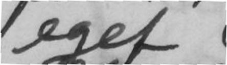eget
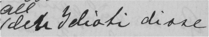det Idioti disse
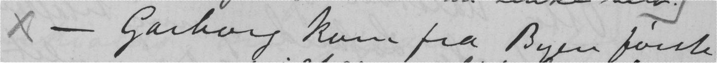x - Garborg kom fra Byen første
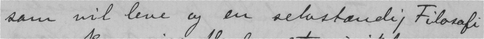som vil leve og en selvstændig Filosofi
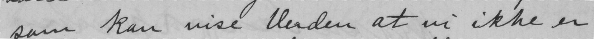som kan vise verden at vi ikke er
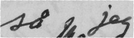så jeg
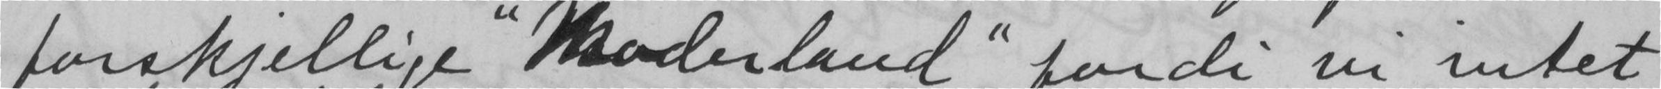forskjellige "moderland" fordi vi intet
